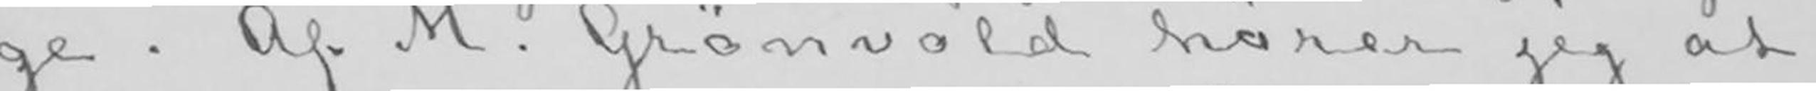ge. Af M. Grønvold hører jeg at
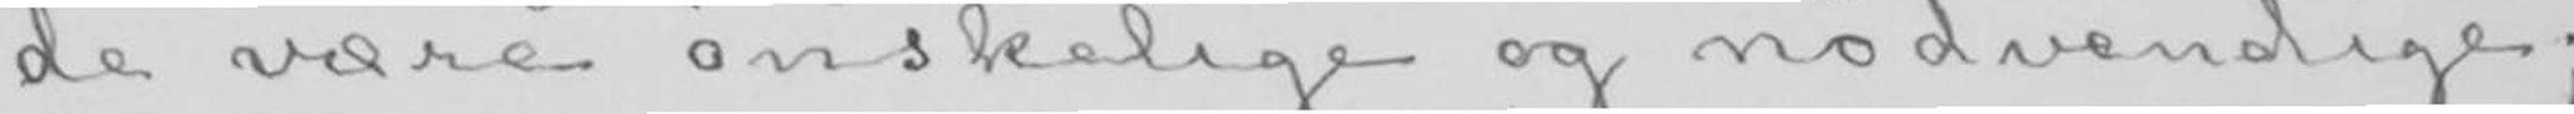de være ønskelige og nødvendige;
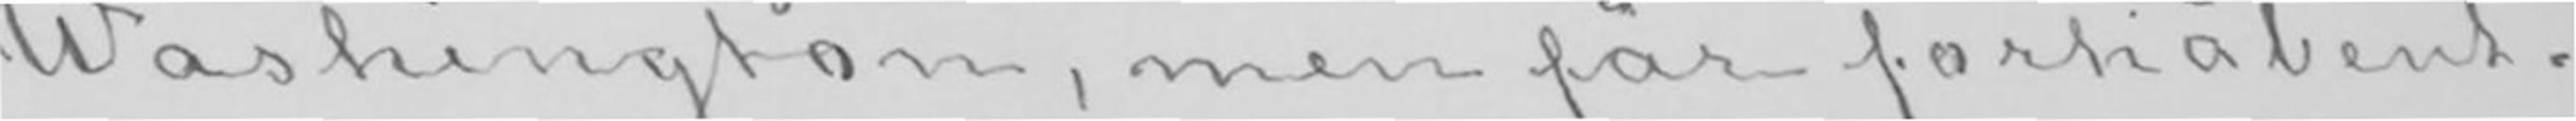Washington, men får forhåbent-
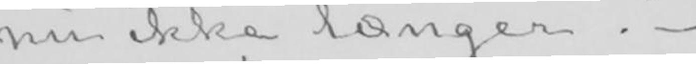nu ikke længer.-
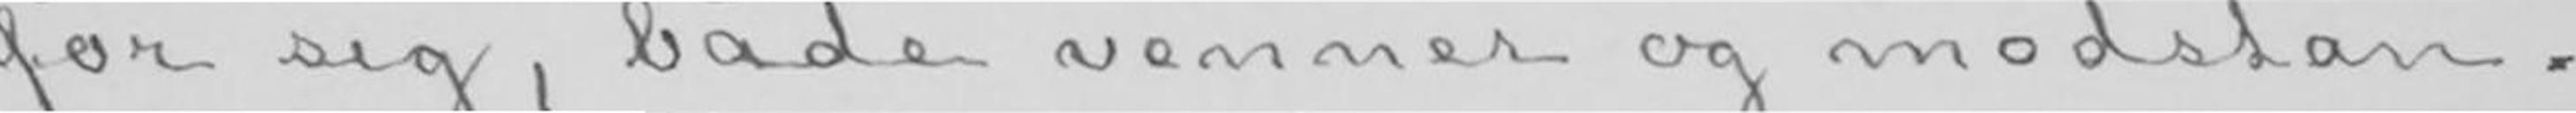for sig, både venner og modstan-
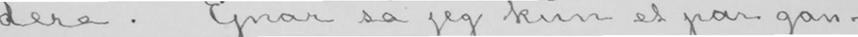dere. Ejnar så jeg kun et par gan-
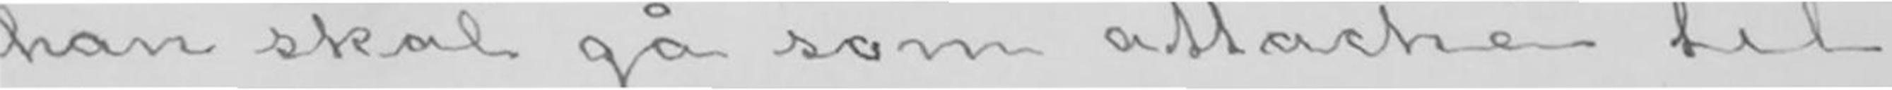han skal gå som attaché til
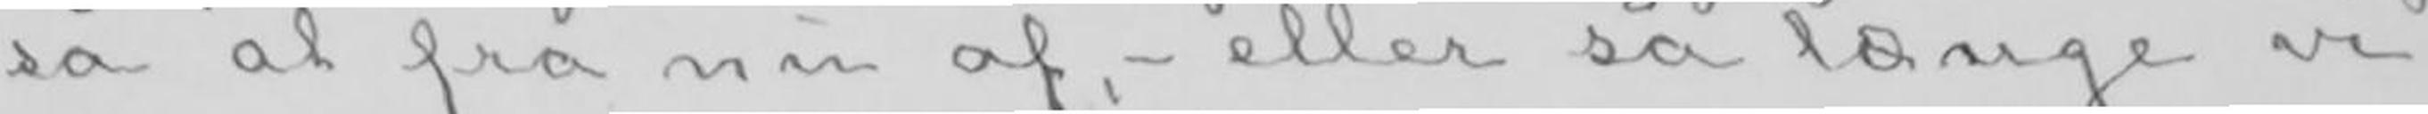så at fra nu af,- eller så længe vi
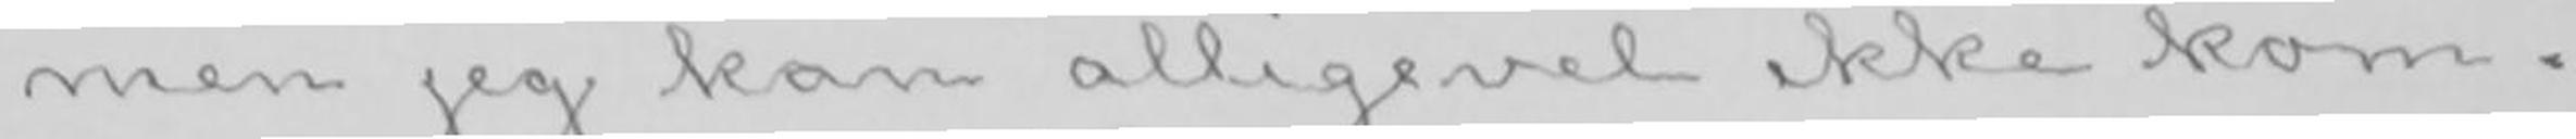men jeg kan alligevel ikke kom-
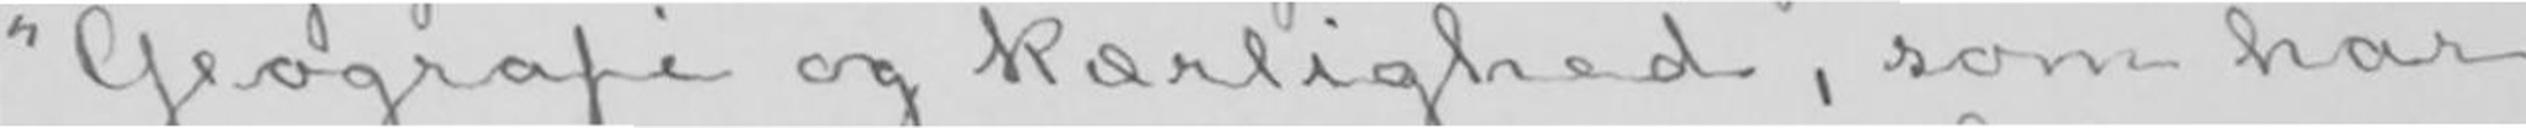«Geografi og kærlighed, som har
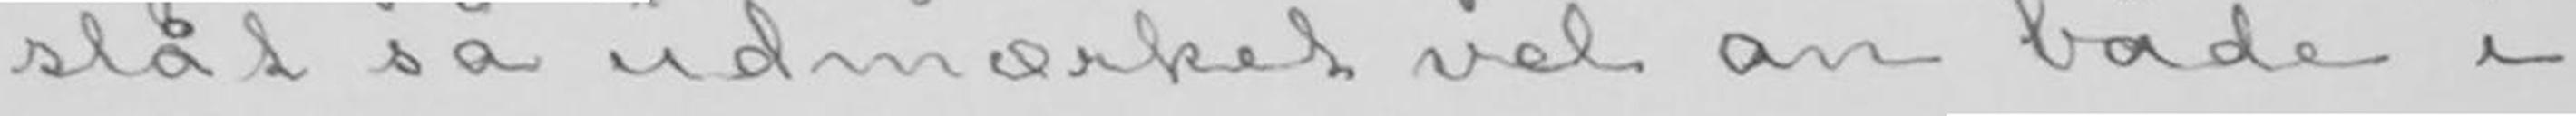slåt så udmærket vel an både i
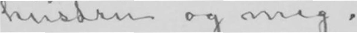hustru og mig.
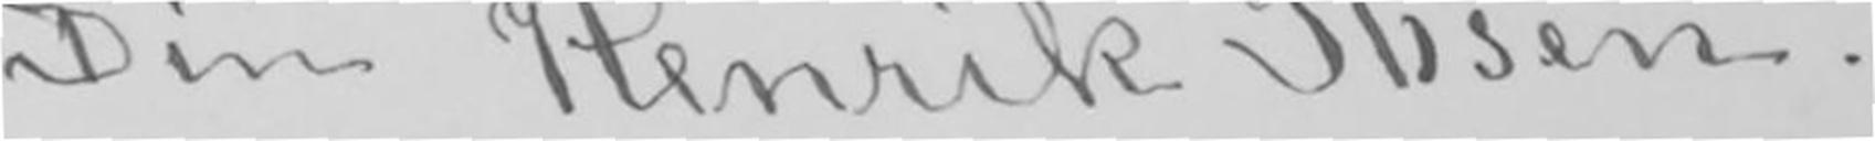Din Henrik Ibsen.
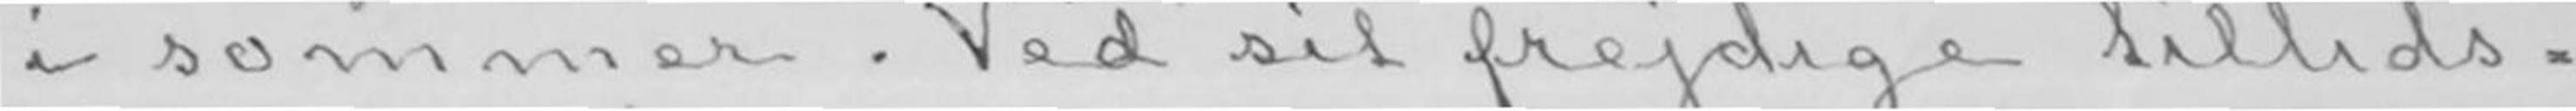i sommer. Ved sit frejdige tillids-
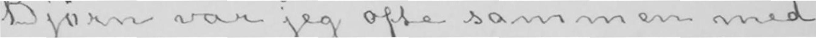Bjørn var jeg ofte sammen med
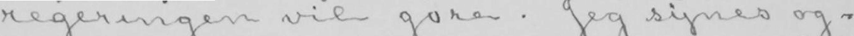regeringen vil gøre. Jeg synes og-
